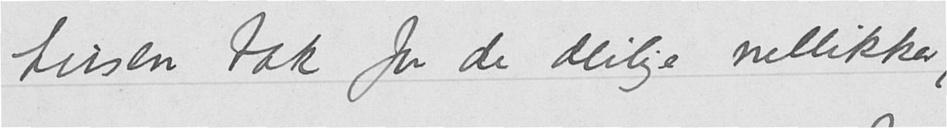tusen tak for de deilige nellikker,
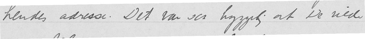hendes adresse. Det var saa hyggelig at De vilde
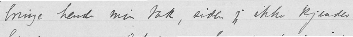bringe hende min tak, siden jeg ikke kjender
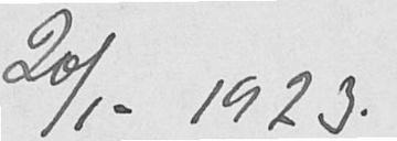20/1-1923.
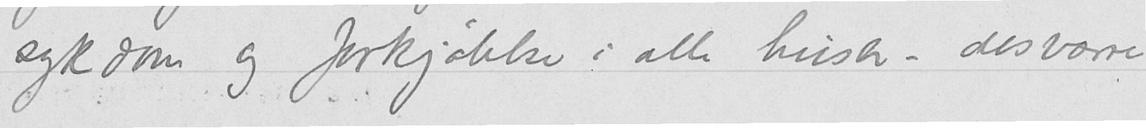sykdom og forkjølelse i alle huser – desværre
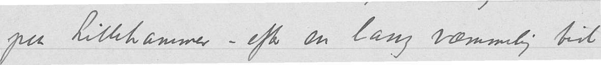paa Lillehammer – efter en lang væmmelig tid
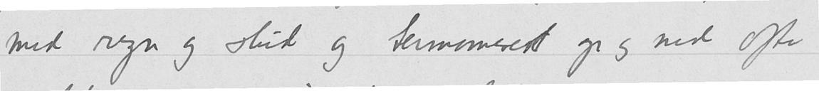med regn og slud og termomeret op og ned ofte
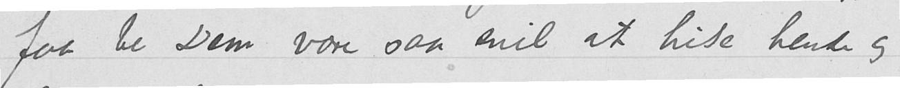faa be Dem være saa snil at hilse hende og
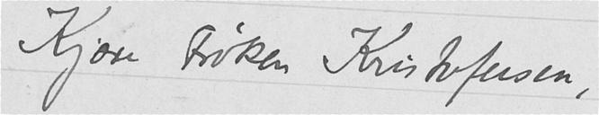Kjære Frøken Kristofersen,
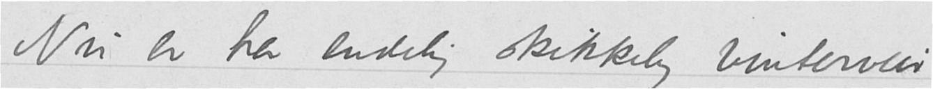Nu er her endelig skikkelig vinterveir
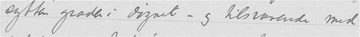sytten grader i døgnet – og tilsvarende med
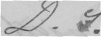D. S.
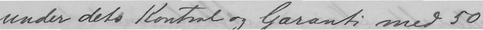under dets Kontrol og Garanti med 50
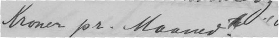Kroner pr. Maaned !
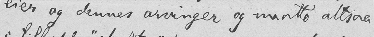eier og dennes arvinger og maatte altsaa
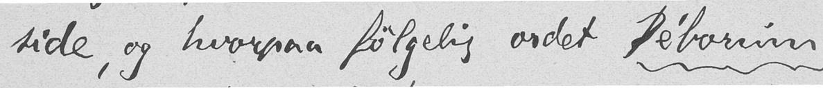side, og hvorpaa følgelig ordet Þéborinn
